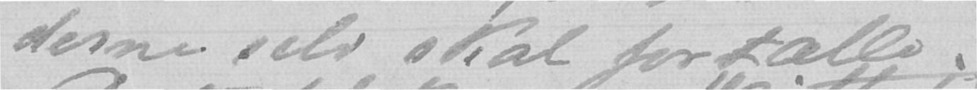derne selv skal fortælle.
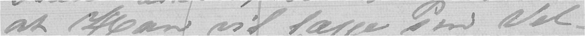at Han vil lægge sin Vel-
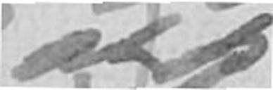at jeg
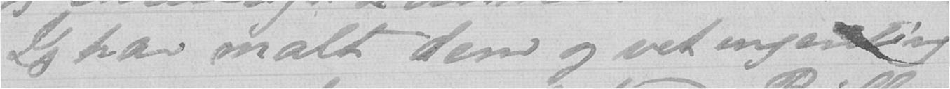Jeg har malt dem og vet ingenting
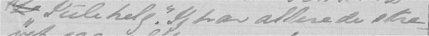H "Julehelg". Jeg har allerede skre-
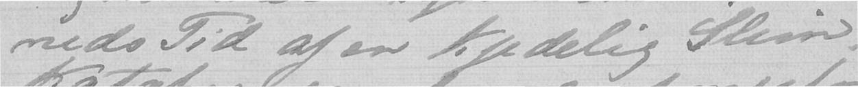neds Tid af en kjedelig Slim-
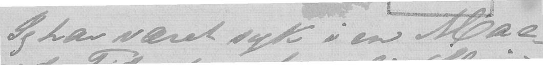Jeg har været syk i en Maa-
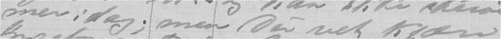mer idag; men Du vet kjære
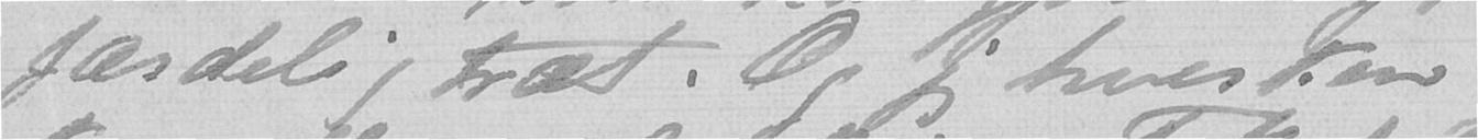færdelig træt. Og jeg hverken
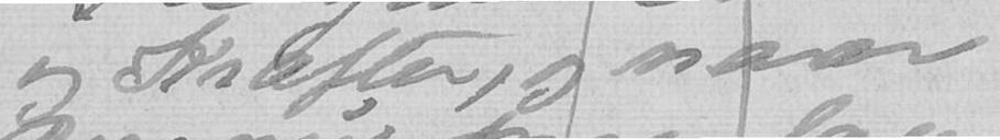og Kræfter, og naar
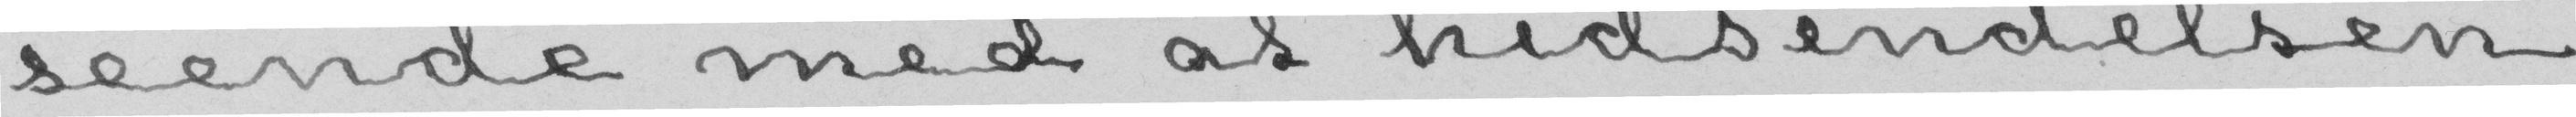seende med at hidsendelsen
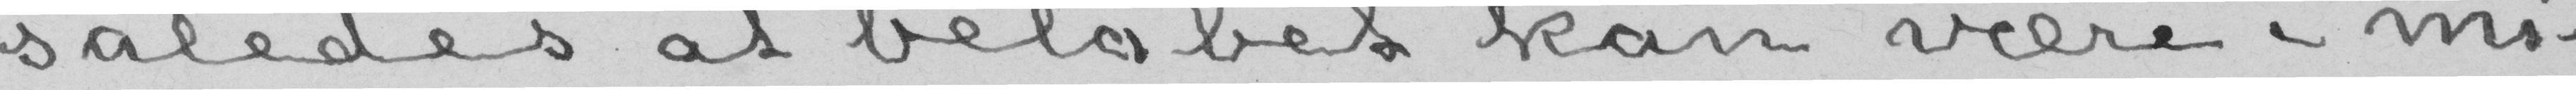således at beløbet kan være i mi-
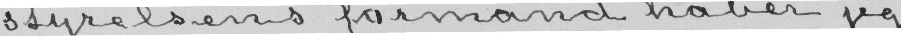styrelsens formand håber jeg
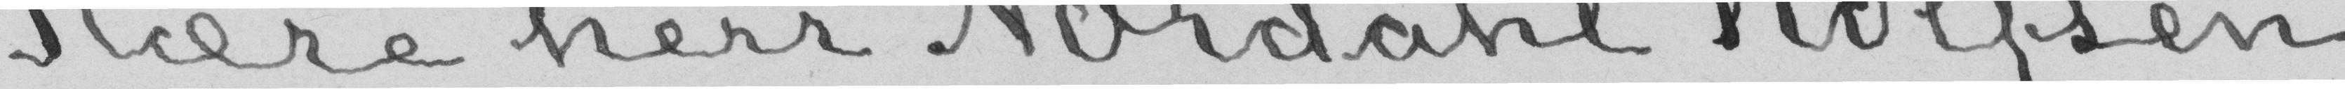Kære herr Nordahl Rolfsen,
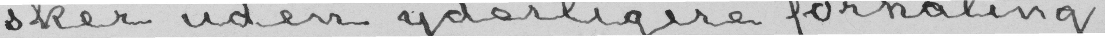sker uden yderligere forhaling,
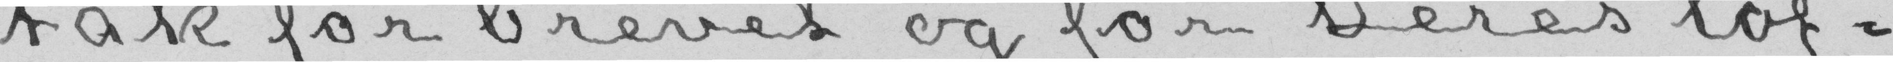tak for brevet og for Deres løf-
